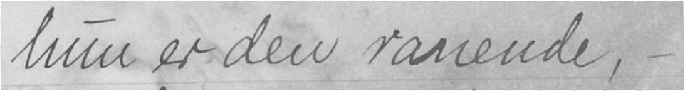hun er den ranende, -
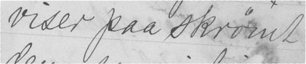viser paa skrømt
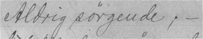Aldrig sørgende; -
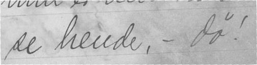se hende, - dø!
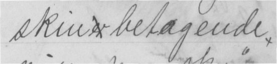skin er betagende,
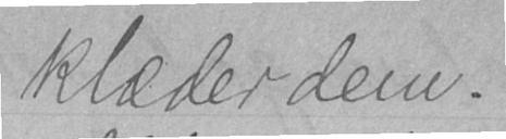klæder dem.
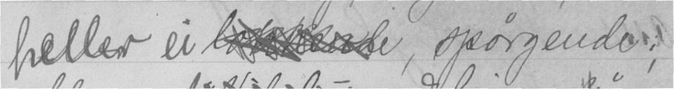heller ei l , spørgende;
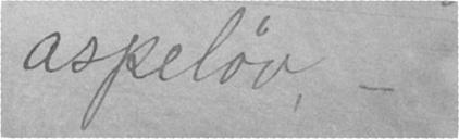aspeløv, -
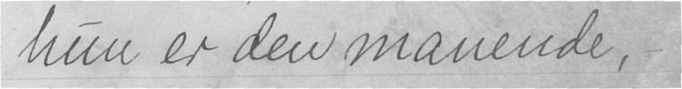hun er den manende, -
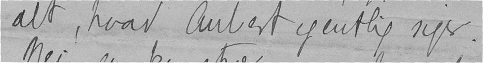alt, hvad Aubert egentlig siger.
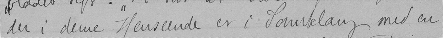der i denne Henseende er i Samklang med en
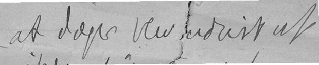at Jæger blev udvist af
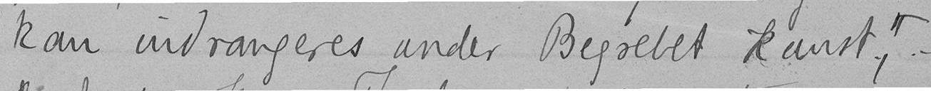kan indrangeres under Begrebet Kunst." -
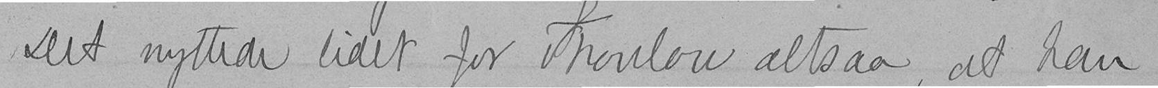Det nyttede lidet for Thaulow altsaa, at han
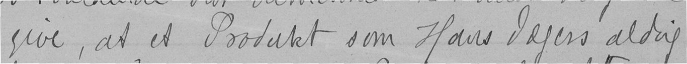give, at et Produkt som Hans Jægers aldrig
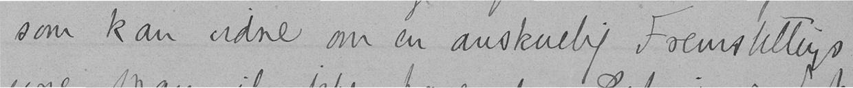som kan vidne om en anskuelig Fremstillings
